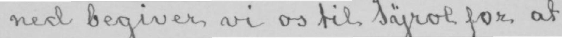ned begiver vi os til Tyrol for at
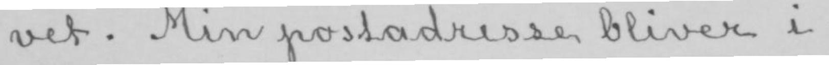vet. Min postadresse bliver i-
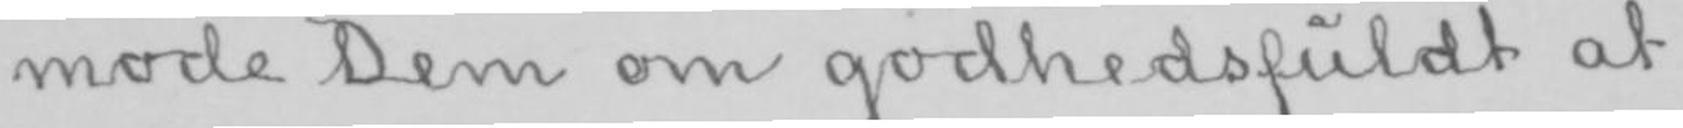mode Dem om godhedsfuldt at
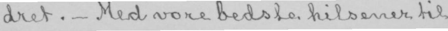dret.- Med vore bedste hilsener til
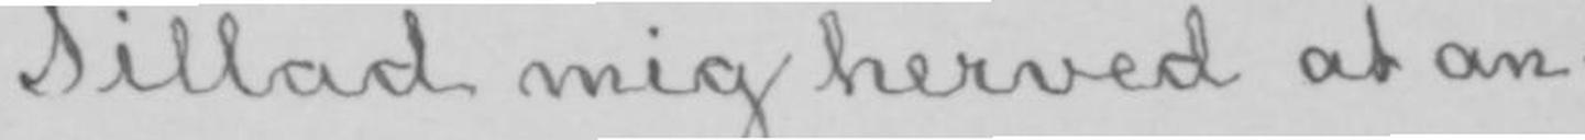Tillad mig herved at an-
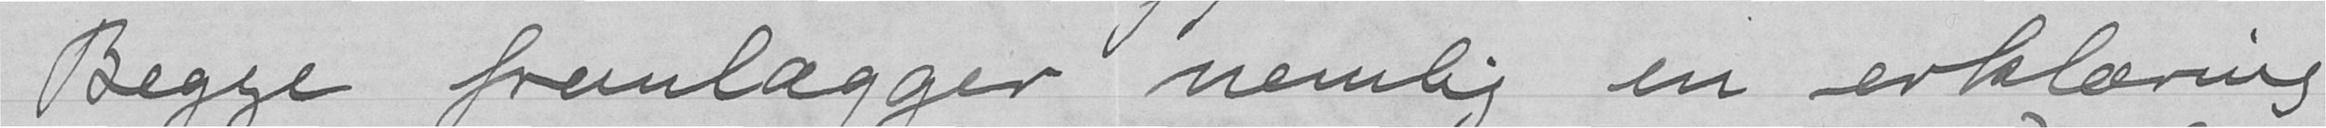Begge fremlægger nemlig en erklæring
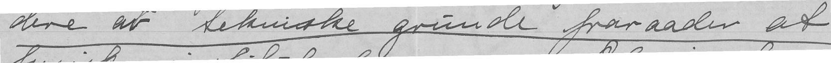dere av tekniske grunde fraraader at
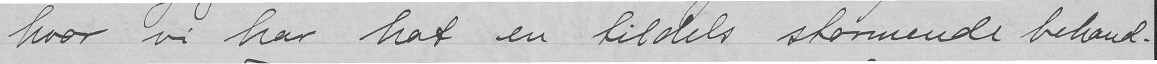hvor vi har hat en tildels stormende behand-
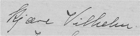Kjære Vilhelm.
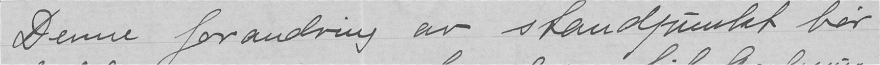Denne forandring av standpunktet bør
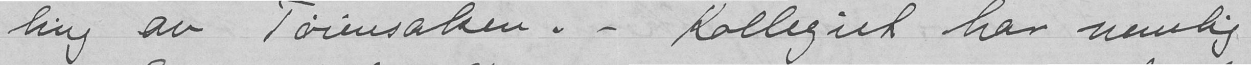ling av Tøiensaken - Kollegiet har nemlig
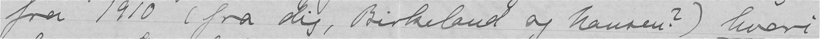fra 1910 (fra dig, Birkeland og hansen?) hvori
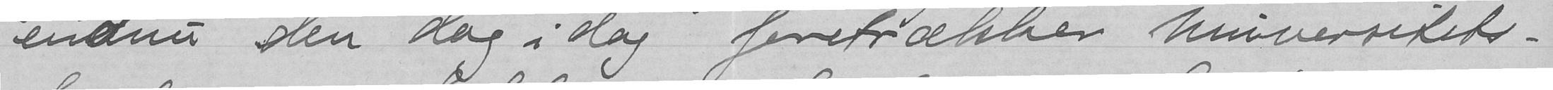endnu den dag i dag foretrækker Unversitets-
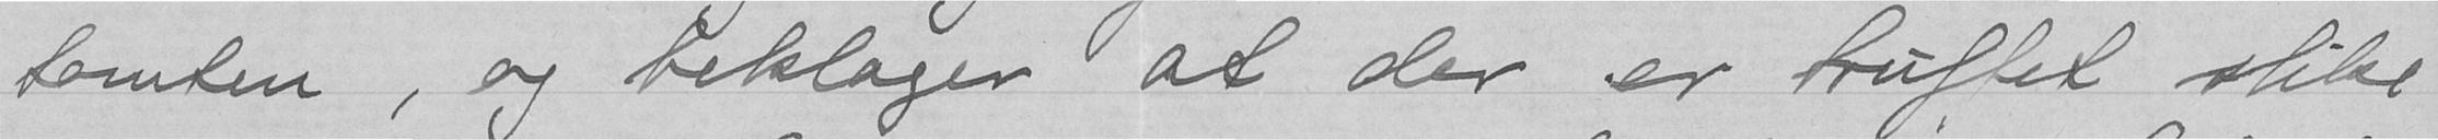tomten, og beklager at der er truffet slike
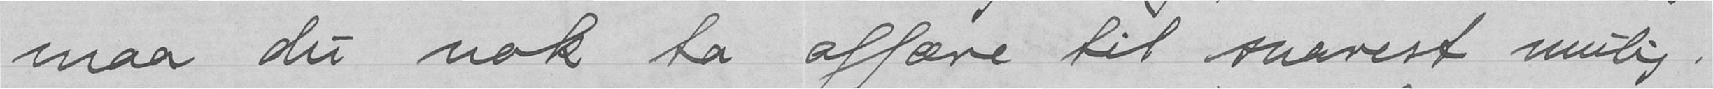maa der nok ta affære til snarest mulig.
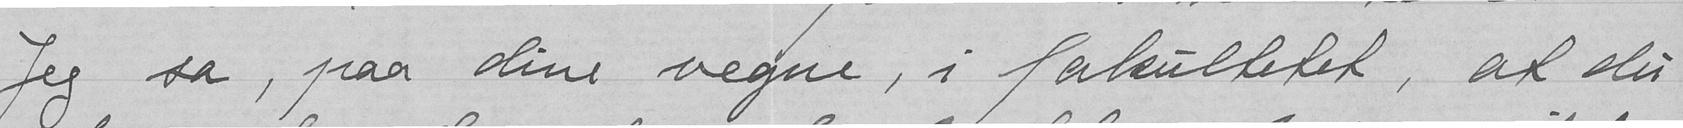Jeg sa, paa dine vegne, i fakultetet, at du
